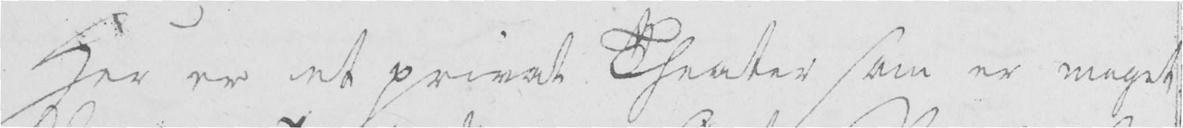Her er et privat Theater som er meget
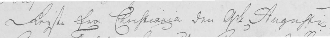Reyste fra Christiania den 9de Augusti;
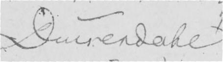Diurendahl
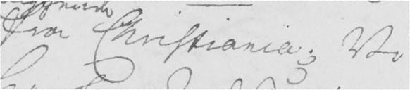fra Christiania; Vi
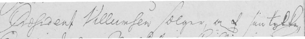Præsident Villumsen sælger af sin tykke,
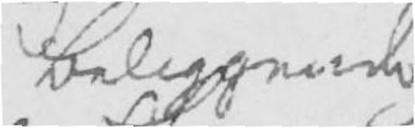beliggende
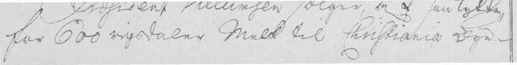for 600 rigsdaler Melk til Christiania Bye -
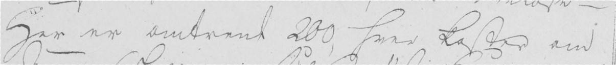Her er omtrent 200, hver koster om
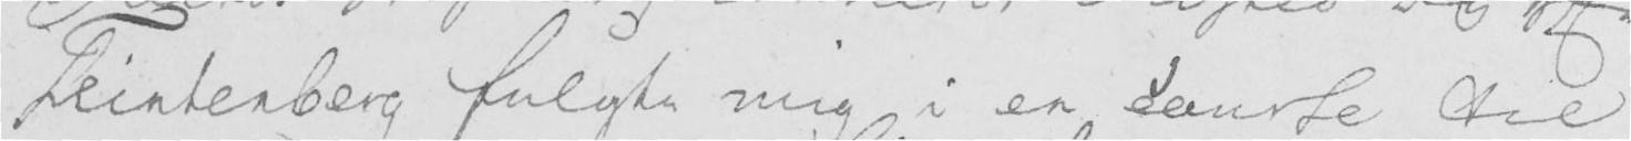Flintenberg fulgte mig i en caurse til
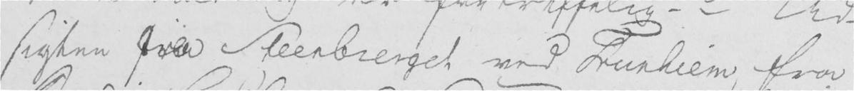sigten fra Steenberget ved Trunhiem, fra
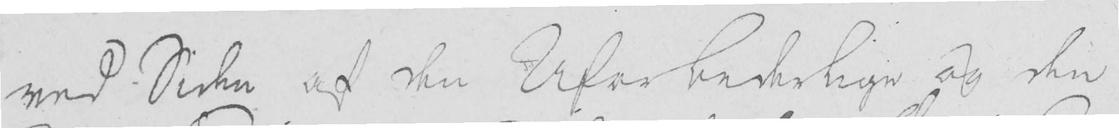ved Siden af den Uforbederlige og den
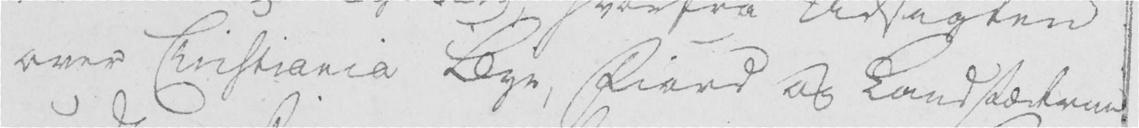over Christiania Bye, Fiord og Landstæderne
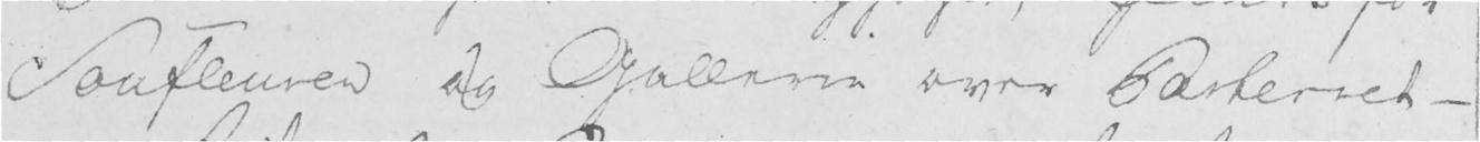Soufleuren og Gallerie over Parterret -
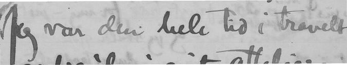Jeg var den hele tid i travelt
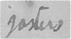Josters
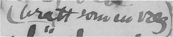(bratt som en væg)
